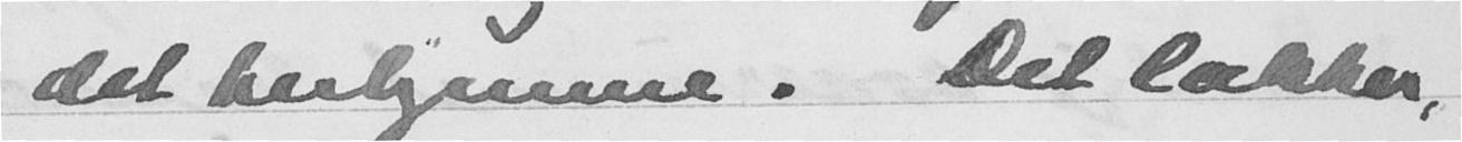det herhjemme. Det lokker,
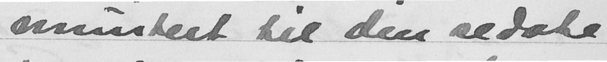muntert til den sedate
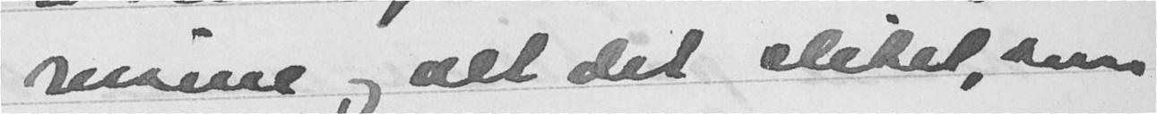eriene og alt det slitet, som
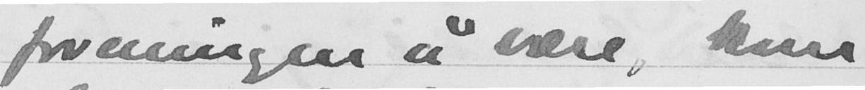foreningen å være, kom
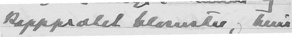kapppratet blomster og hun
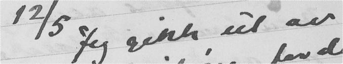12/5 Jeg gikk ut av
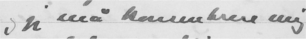og jeg må konsentrere mig
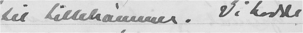til Lillehammer. Vi hadde
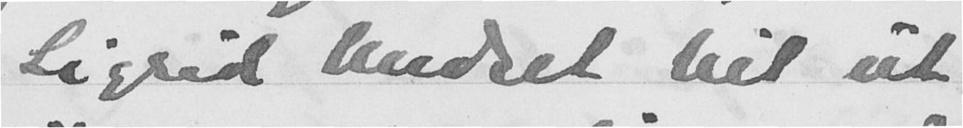Sigrid Undset hit ut
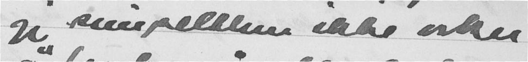jeg simpelthen ikke orker
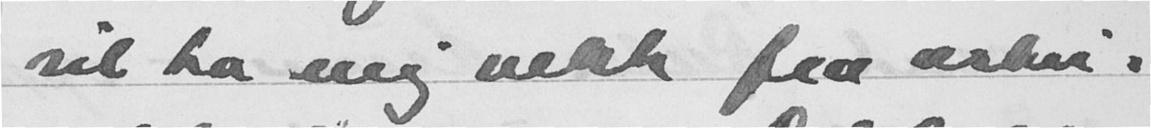vil ha mig vekk fra arbei-
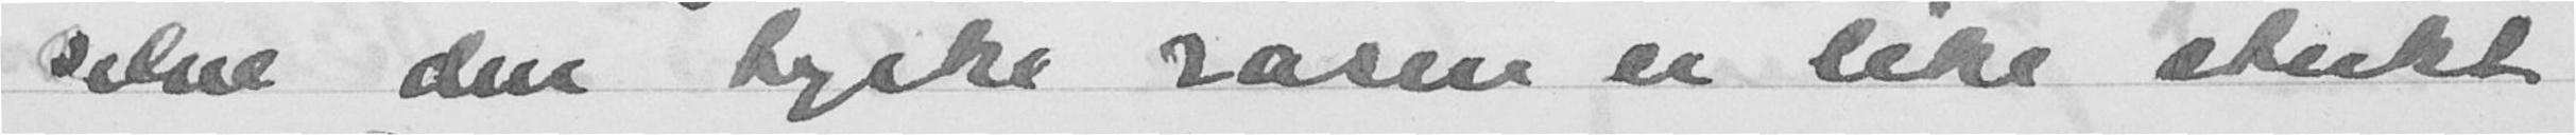selve den tyske rasen er like sterkt
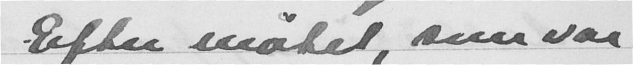Efter møtet, som var
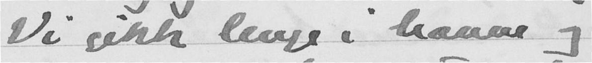Vi gikk lenge i haven og
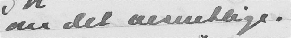om det vesentlige.
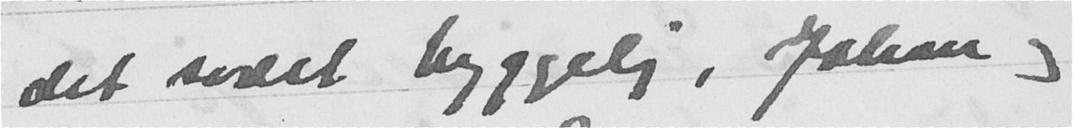det svært hyggelig, Johan og
